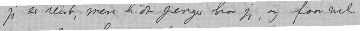jeg er reist, men lidt penge har jeg, og faar vel
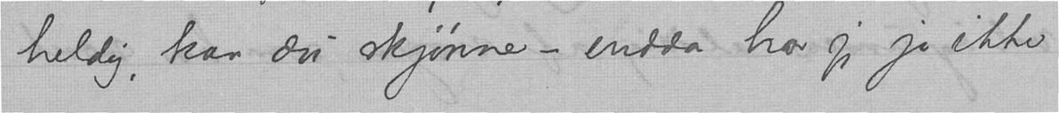heldig, kan du skjønne - endda har jeg jo ikke
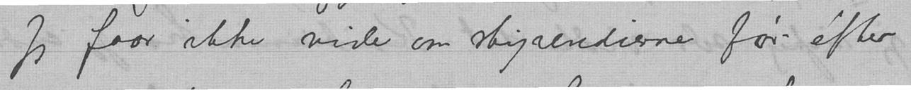Jeg faar ikke vide om stipendierne før efter
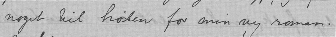noget til høsten for min ny roman.
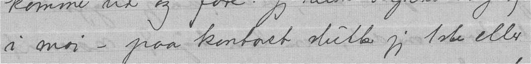i mai - paa kontoret slutter jeg 1ste eller.
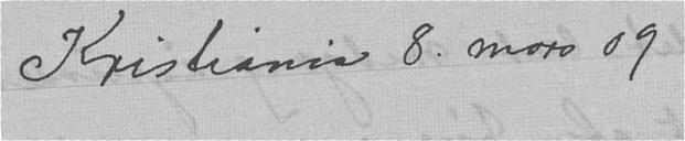Kristiania 8. mars 09
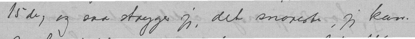15de, og saa stryger jeg, det snareste, jeg kan.
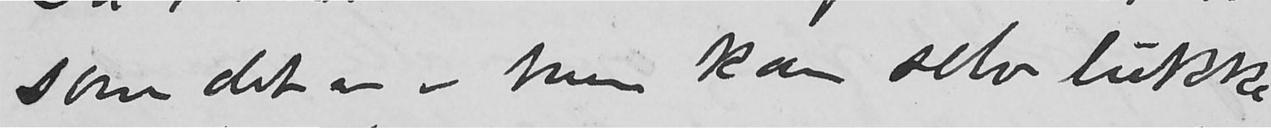som det er - hun kan selv lukke
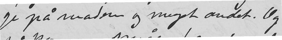ge på maden og meget andet. Og
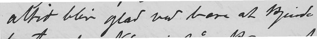altid blir glad ved bare at kjende
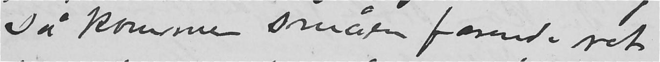så kommer småen farende ret
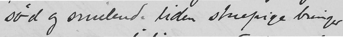sød og smilende liden stuepige bringer
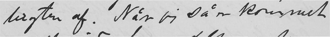lugten af. Når jeg så er kommet
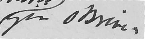gir skrive-
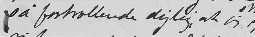så fortrollende dejlig, at jeg
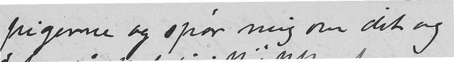pigerne og spør mig om dit og
